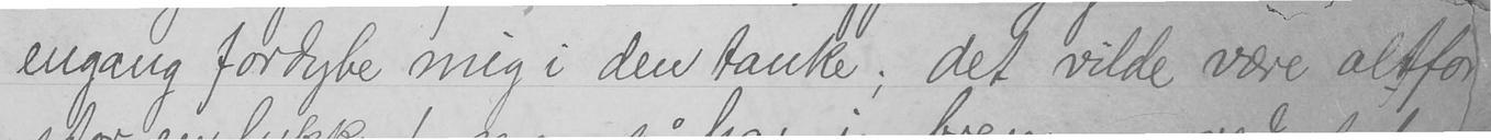engang fordybe mig i den tanke; det vilde være altfor
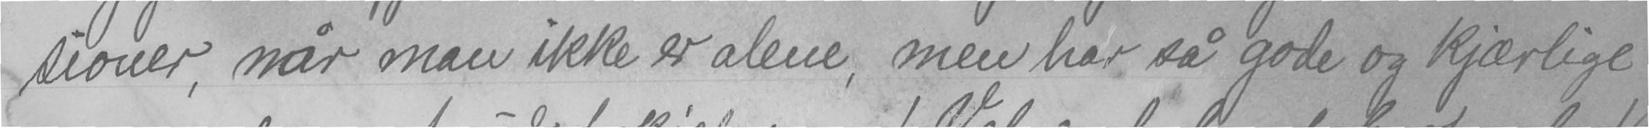sioner, når man ikke er alene, men har så gode og kjærlige
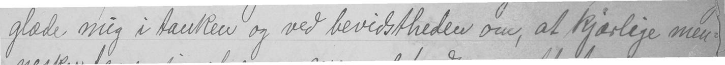glæde mig i tanken og ved bevidstheden om, at kjærlige men-
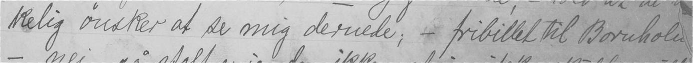kelig ønsker at se mig dernede, - fribillet til Bornholm
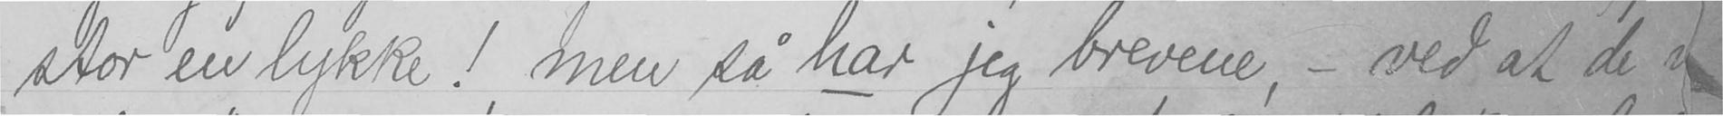stor en lykke! men så har jeg brevene, - ved at de vir-
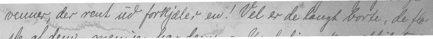venner, der rent ud forkjæler en! Vel er de langt borte, de fle-
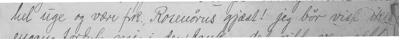hel uge og være frk. Rosenørns gjæst! jeg bør vist ikke
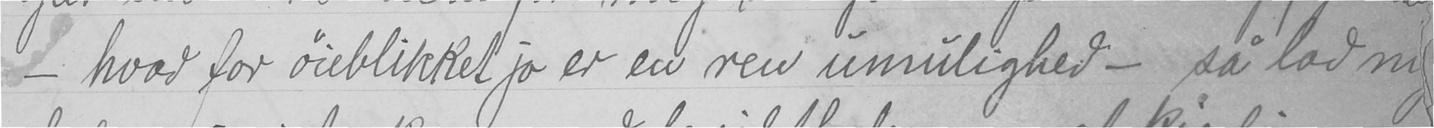- hvad for øiblikket jo er en ren umulighed - så lad mig
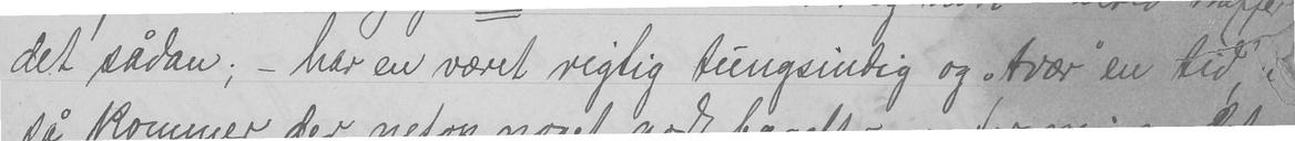det sådan; - har en været riglig tungsindig og "tvær" en tid,
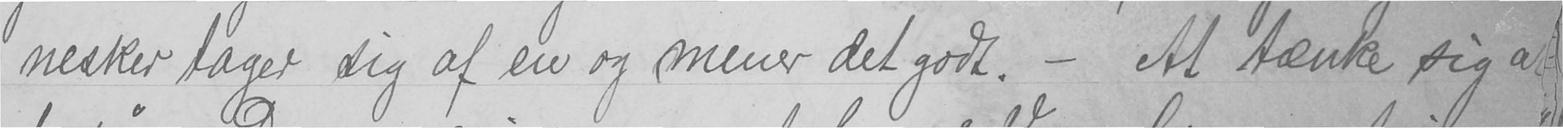nesker tager sig af en og mener det godt. - At tænke sig at
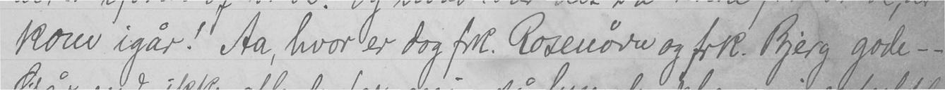kom igår! Aa, hvor er dog frk. Rosenørn og frk. Bjerg gode - -
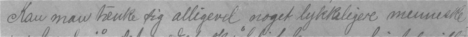Kan man tænke sig alligevel noget lykkeligere menneske
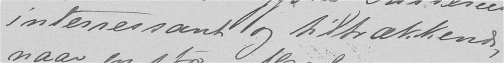interessant og tiltrækkende,
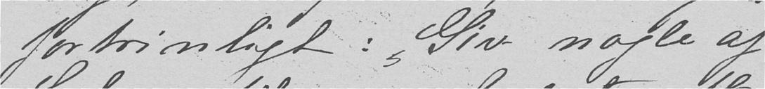fortrinligt. Giv nogle af
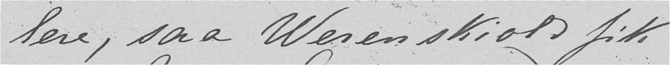lere, saa Werenskiold fik
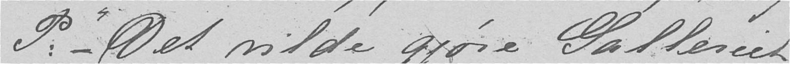P: - Det vilde gjøre Galleriet
